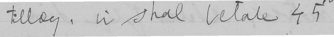tillæg, vi skal betale 45
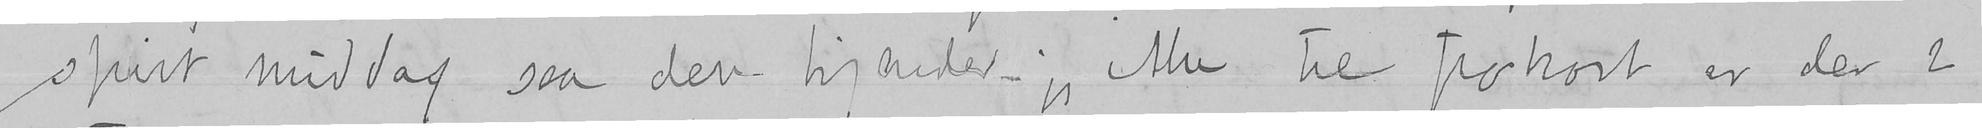spist middag, saa den kjender jeg ikke til frokost er der 2
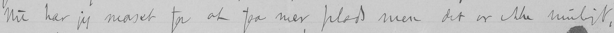Nu har jeg maset for at faa mer plads men det er ikke muligt,
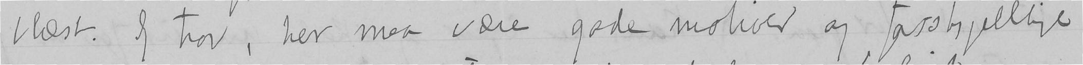blæst. Jeg tror, her maa være gode motiver og forskjelligt
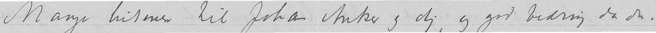Mange hilsener til Johan Anker og dig, og god bedring da du!
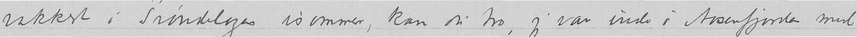vakkert i Trøndelagen isommer, kan du tro, jeg var inde i Aasenfjorden med
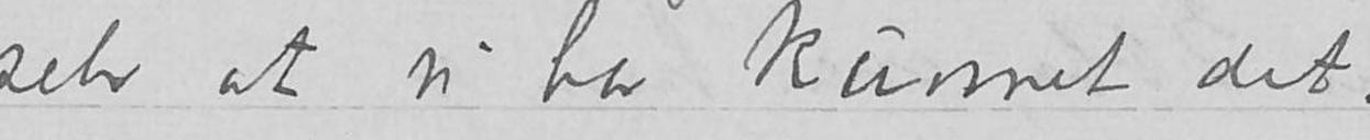selv at vi har kunnet det.
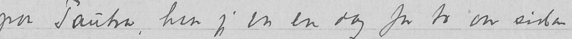stranden paa Tautra, hvor jeg var en dag for to aar siden.
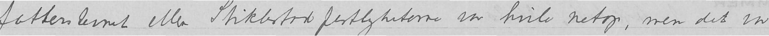fatterstevnet eller Stiklestadfestligheterne var hvile netop, men det var
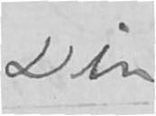Din
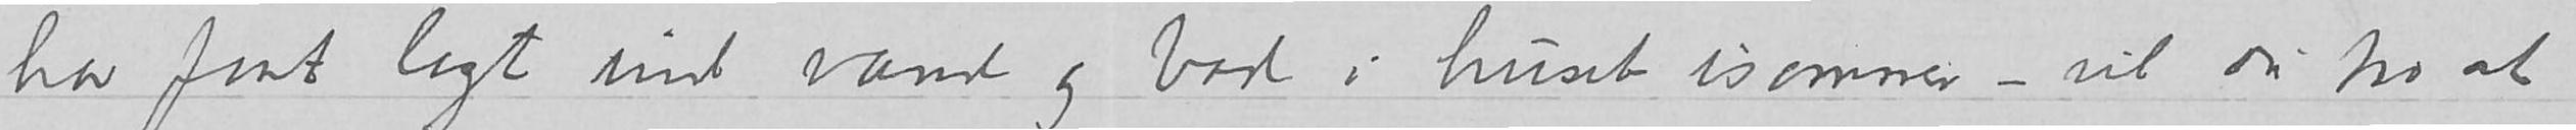Jeg har faat lagt ind vand og bad i huset isommer – vil du tro at
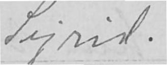Sigrid
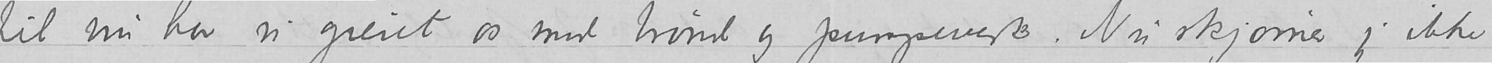til nu har vi greiet os med brønd og pumpeverk. Nu skjønner jeg ikke
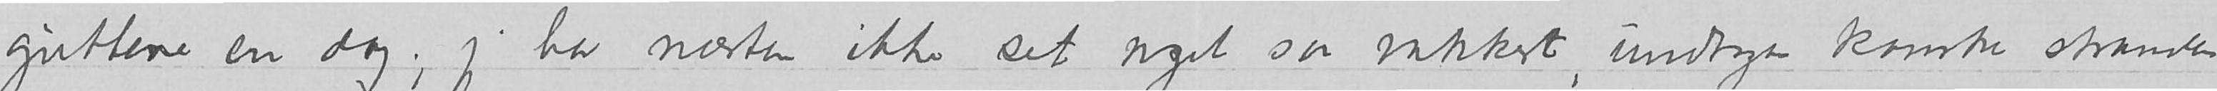guttene en dag; jeg har næsten ikke set noget saa vakkert, undtagen kanske stranden
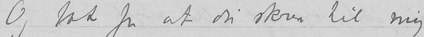Og tak for at du skrev til mig
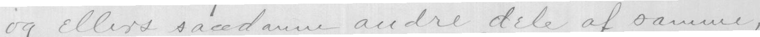og Ellers saadanne andre dele af samme,
